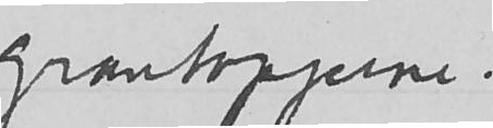grantopperne.
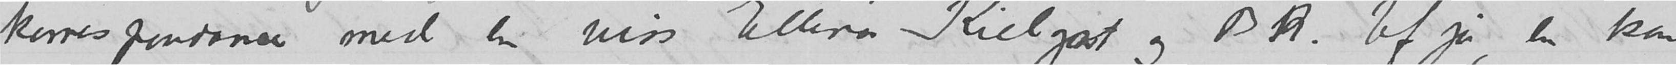korrespondanse med en viss Ellinor Kielgast  og BR. Uf ja, en kan
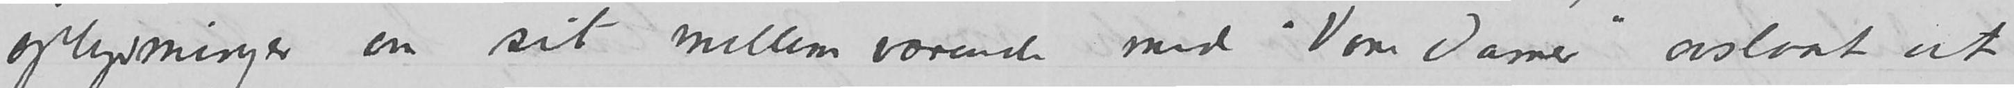oplysninger om sit mellemværende med «Voaare Damer» avslaat at
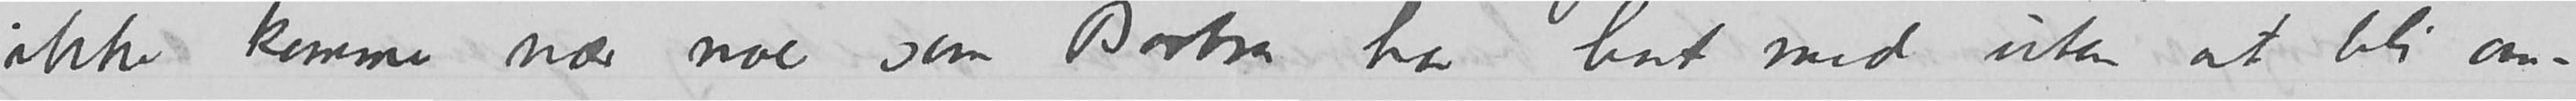ikke komme nær noe som Barbra har hat med uten at bli om-
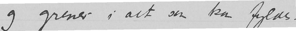og grener i alt som kan fyldes.
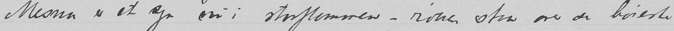Mesna er et syn nu i storflommen – røken staar over de høieste
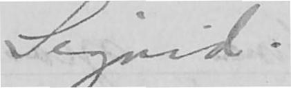Sigrid
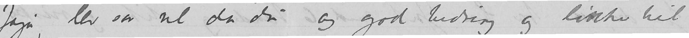Jaja, lev saa vel da du og god bedring og lykke til
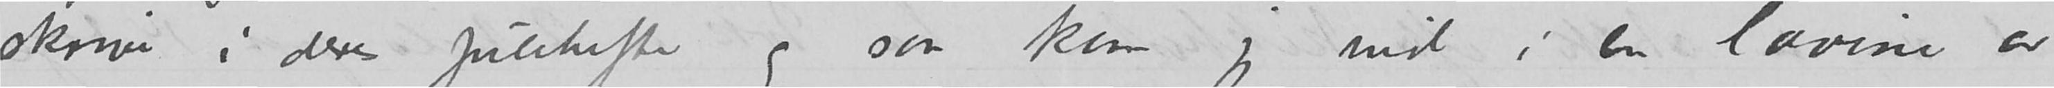skrive i deres julehefte og saa kom jeg midt i en lavine av
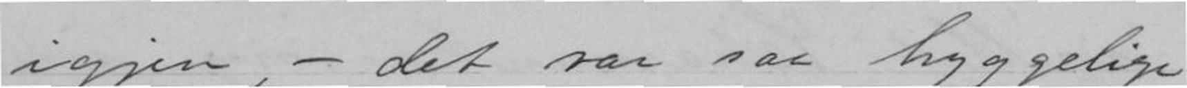igjen, - det var saa hyggelige
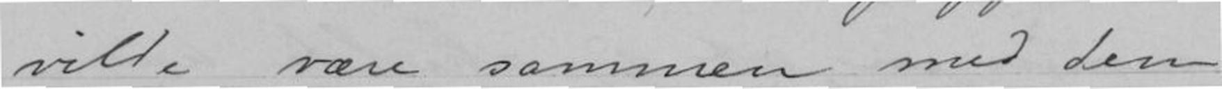vilde være sammen med dem
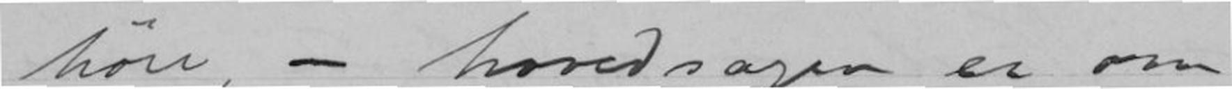høre, - hovedsagen er om
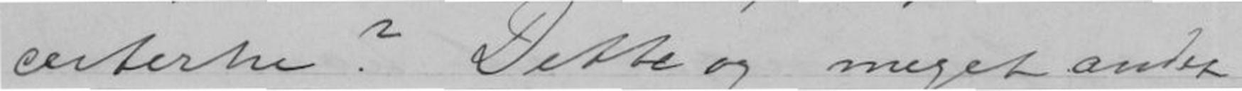certerne? Dette og meget andet
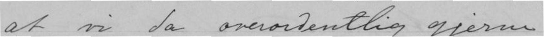at vi da overordentlig gjerne
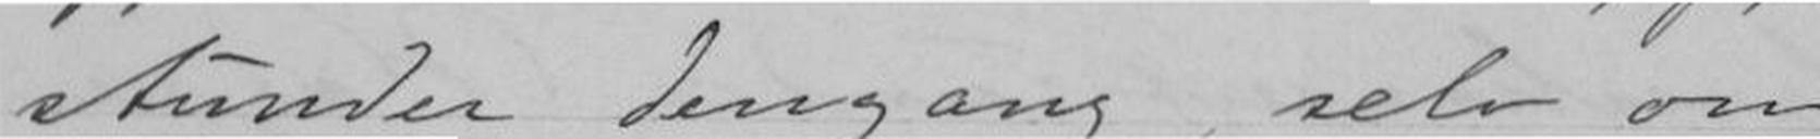stunder dengang, selv om
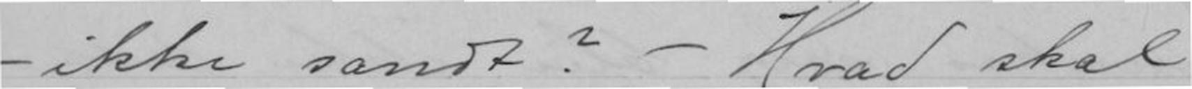- ikke sandt? - Hvad skal
